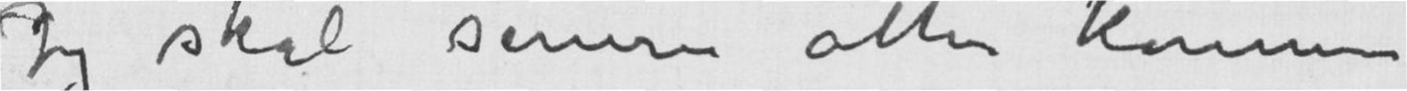Jeg skal senere atter komme
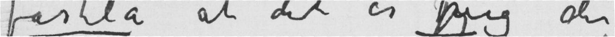fastslå at det er jmig der
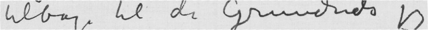tilbage til de Grundrids jeg
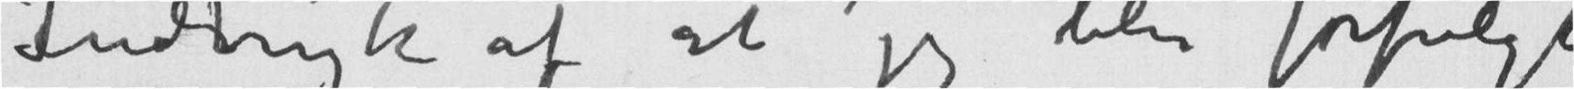iIndtryk af at jeg blir forfulgt
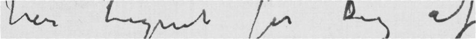har tegnet for Dig af
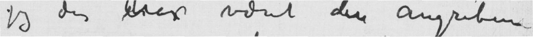jeg der erhar været den angribene
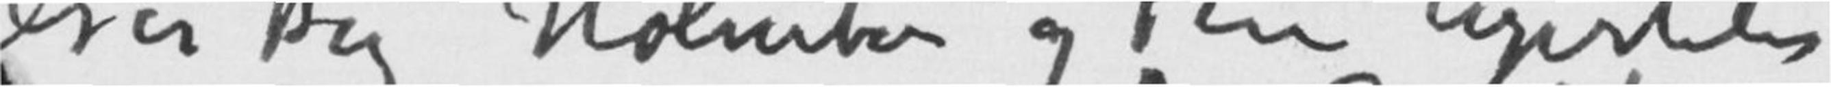lser Dig Holmboe og Frue hjerteli
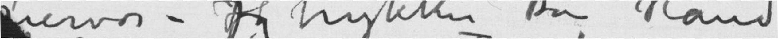nervøs – Jeg trykker Din Hånd
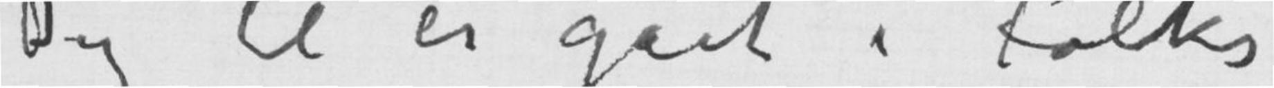Dig til er gået i Folks
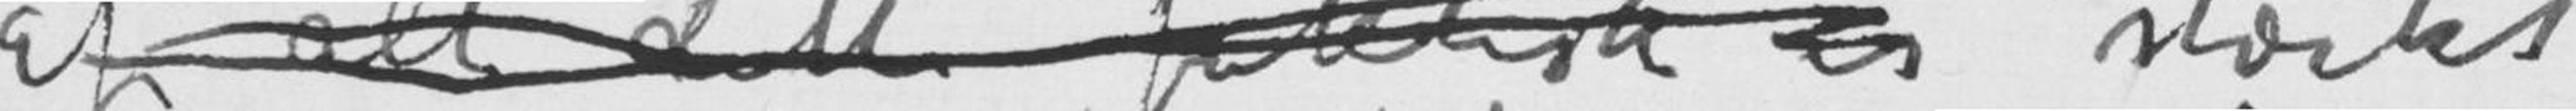af alt dette faktisk er stærkt
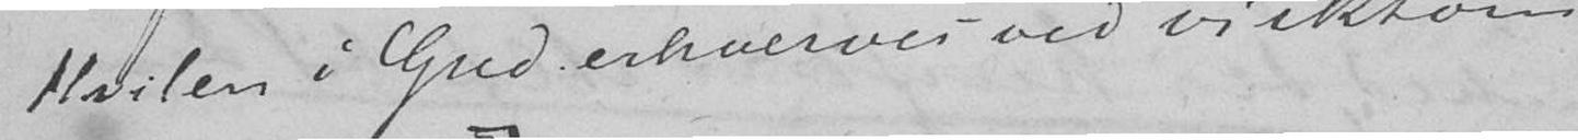Hvilen i Gud erhverves ved virksom
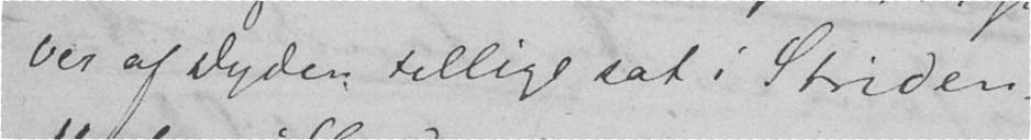ver af Dyden tillige sat i Striden.
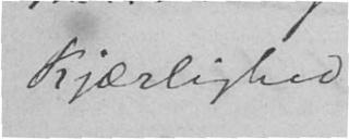Kjærlighed]
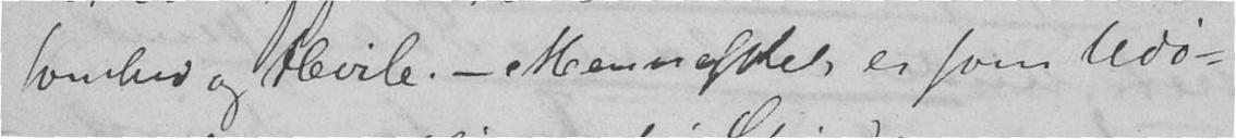somhed og Hvile. – Mennesket er som Udø-
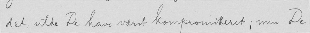det, vilde De have været kompromitteret; men De
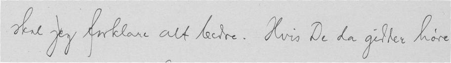skal jeg forklare alt bedre. Hvis De da gidder høre
